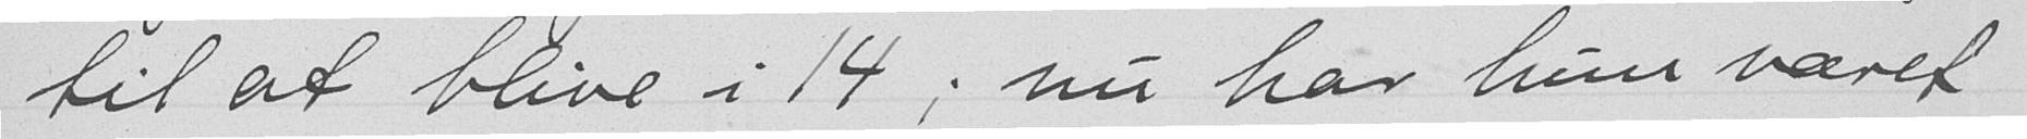til at blive i 14; nu har hun været
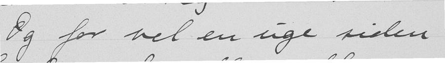Og for vel en uge siden
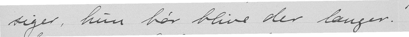siger, hun bør blive der længer.
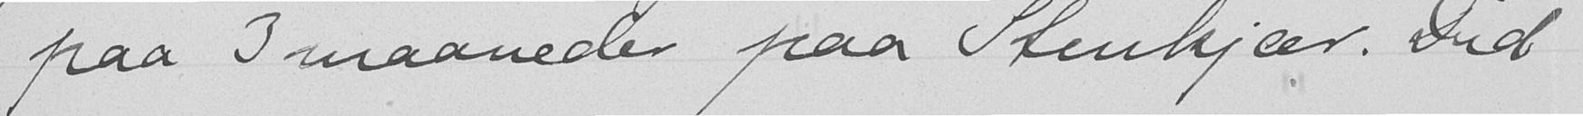paa 3 maaneder paa Stenkjær. Did
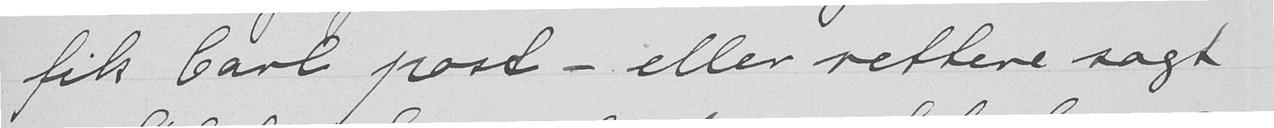fik Carl post - eller rettere sagt
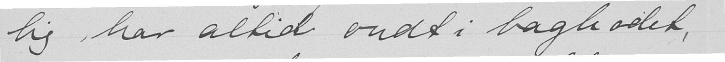lig, har altid ondt i baghodet,
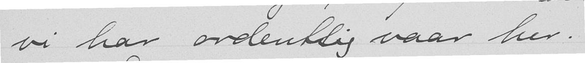vi har ordentlig vaar her.
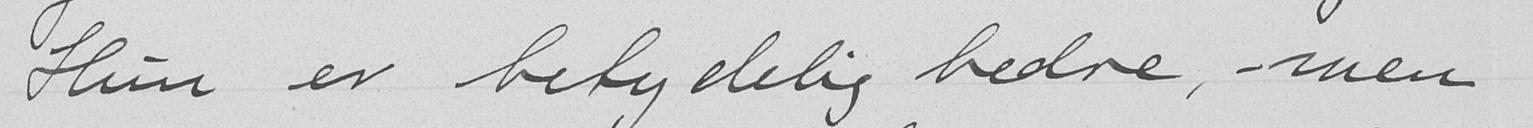Hun er betydelig bedre, - men
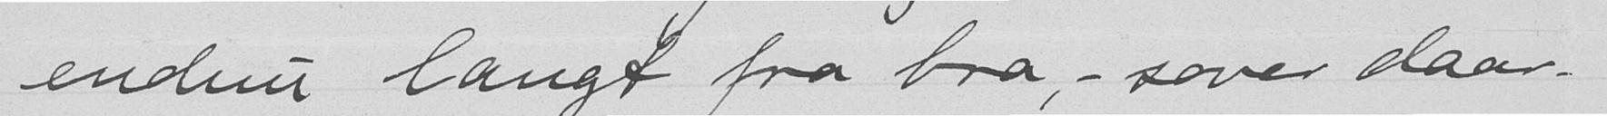endnu langt fra bra, - sover daar-
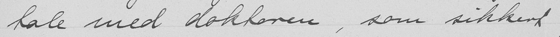tale med doktoren, som sikkert
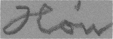Høn
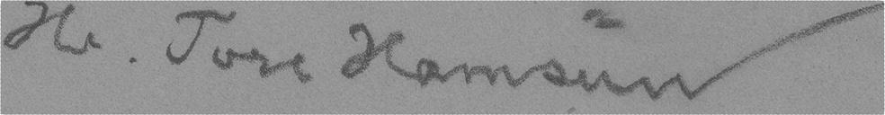Hr. Tore Hamsun
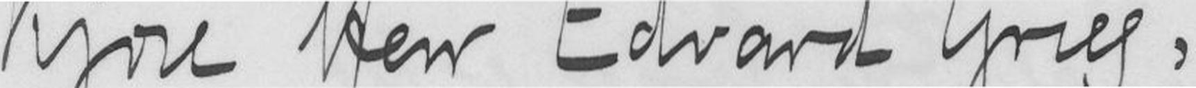Kjære Herr Edvard Grieg,
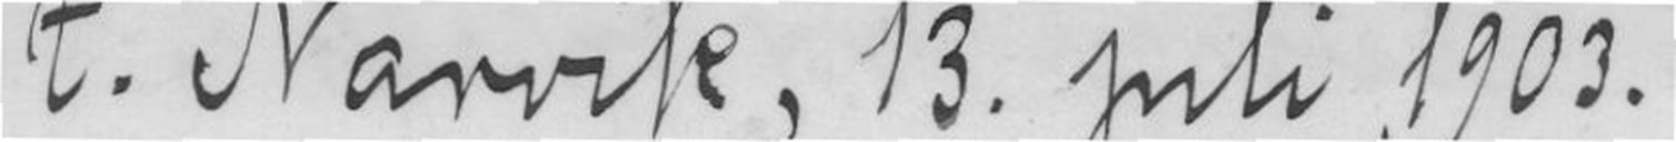t. Narvik, 13 juli 1903.
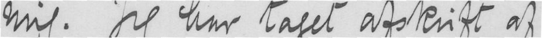mig. Jeg har taget afskrift af
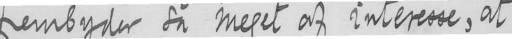frembyder så meget af intersse, at
